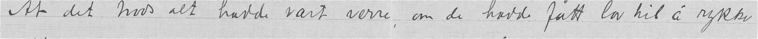At det trods alt havde vært verre, om de hadde fått lov til å rykke
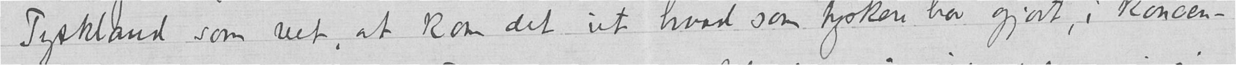Tyskland som vet, at kom det ut hvad som tyskere har gjort, i koncen-
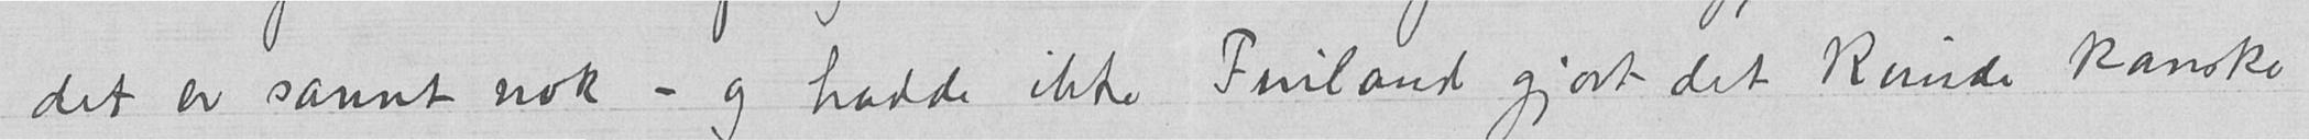det er sannt nok – og hadde ikke Finland gjort det kunde kanske
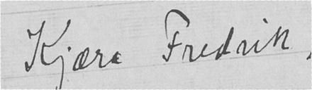Kjære Fredrik,
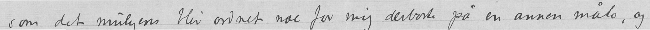som det muligens blir ordnet noe for mig derborte på en annen måte, og
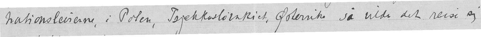trationsleirerne, i Polen, Tsjekkoslovakiet, Østerrike sa vilde det reise sig
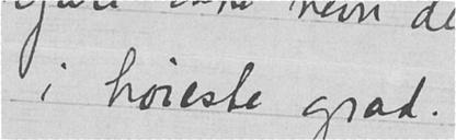i høieste grad.
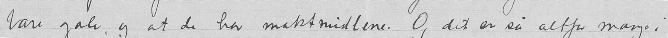bare gale, og at de har maktmidlene. Og det er jo altfor mange i
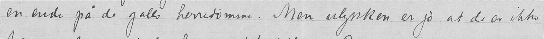en ende pa de gales herredømme. Men ulykken er jo at de er ikke
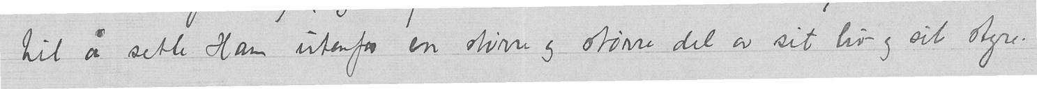til å sette Ham utenfor en større og større del av sit liv og sit styre.
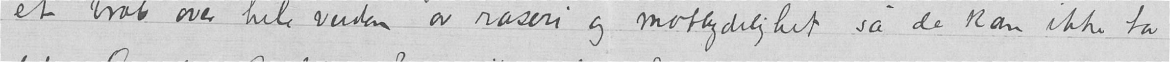et brøl over hele verden av raseri og motbydelighet så de kan ikke ta
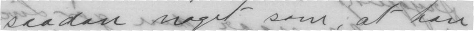saadan noget som, af han
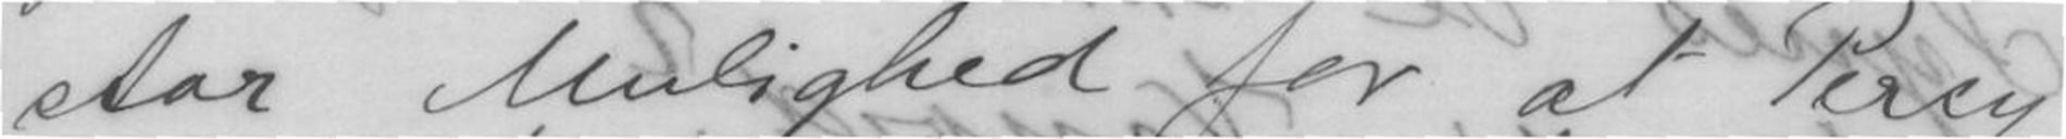stor Mulighed for at Percy
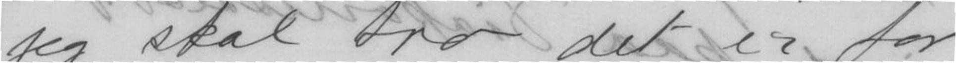jeg skal tro det er for
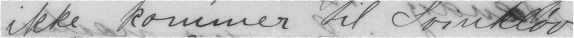ikke kommer til Svinkløv.
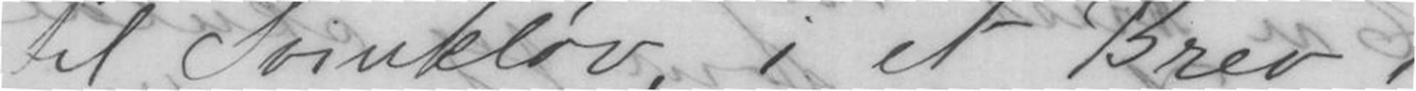til Svinkløv, i et Brev i
